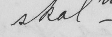skal
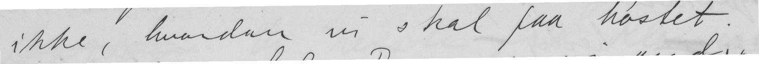ikke, hvordan vi skal faa høstet.
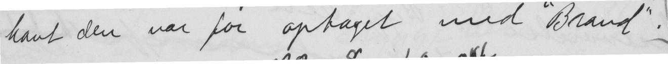havt den var for optaget med "Brand".
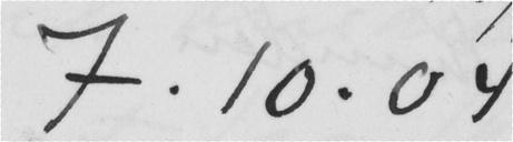7.10.04
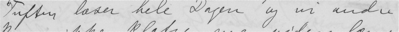Tuften læser hele Dagen og vi andre
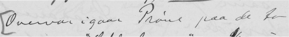Overvar igaar Prøve paa de to
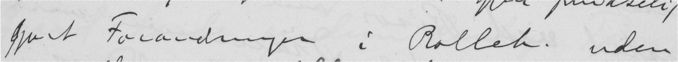gjort Forandringer i Rolleb. uden
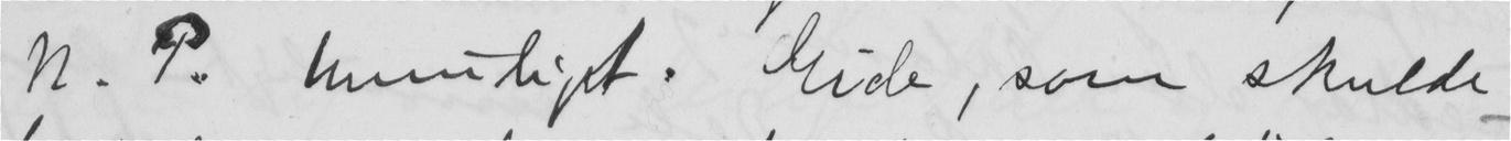N P. umuliget. Eide, som skulde
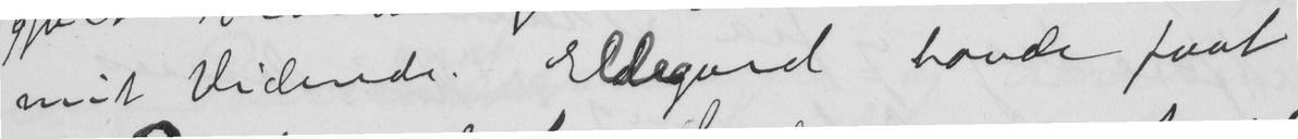mit Vidende. Eldegard havde faat
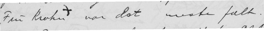Fru Krohn var det meste fælt.
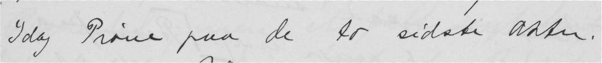Idag Prøve paa de to sidste Akter.
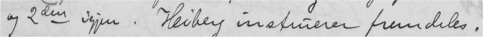og 2den igjen. Heiberg instruerer fremdeles.
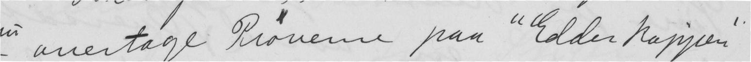overtage Prøverne paa "Edderoppen"
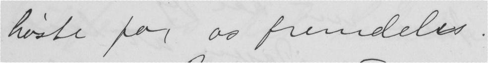høste for os fremdeles.
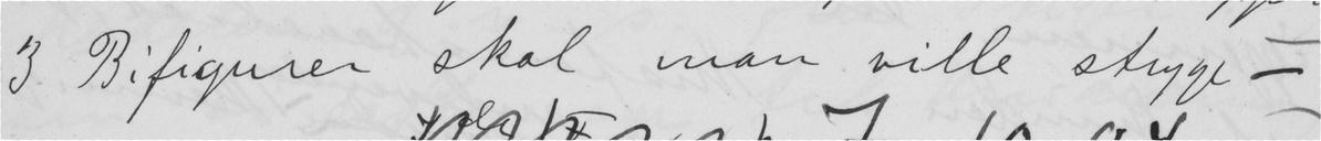3 Bifigurer skal man ville stryge -
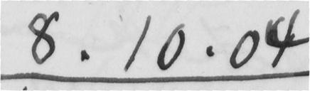8.10.04
